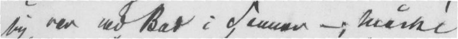jeg var bad i Sommer -; måske
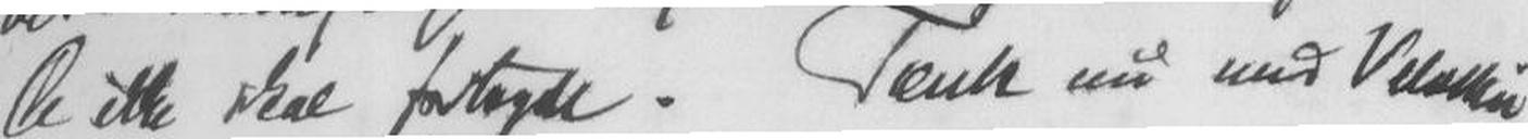De ikke skal fort.Tenk nu med V
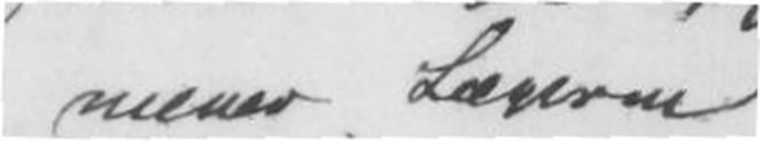mener Lægerne
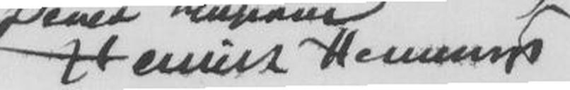Henrik Hennings
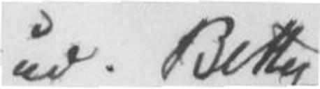ud. Betty
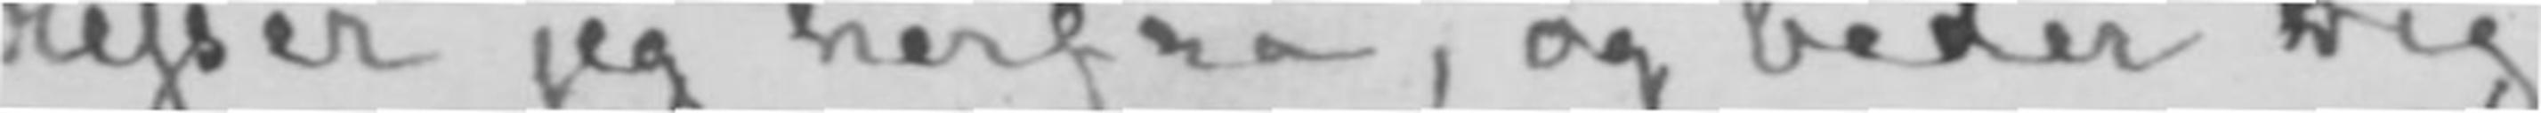rejser jeg herfra, og beder Dig,
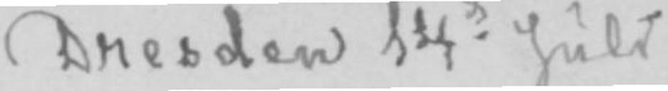Dresden 14de Juli
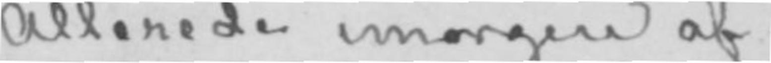Allerede imorgen af-
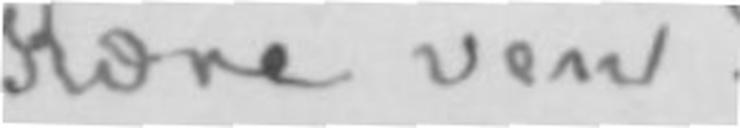Kære ven!
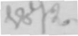1872-
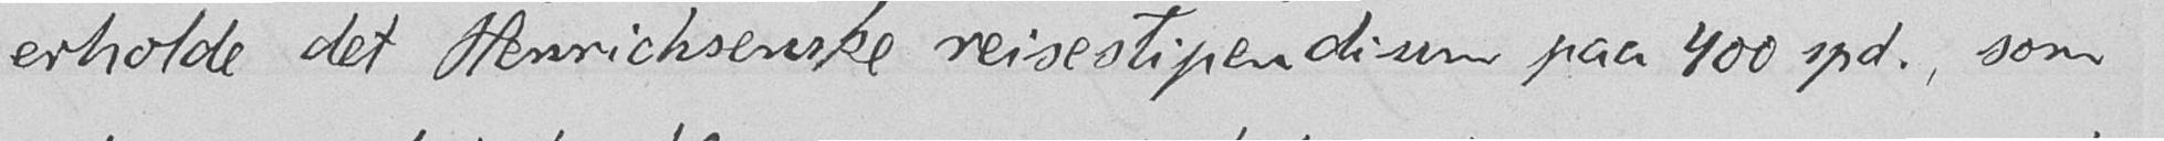erholde det Henrichsenske reisestipendium paa 400 spd., som
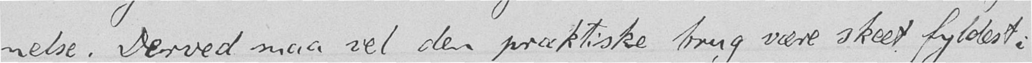nelse. Derved maa vel den praktiske brug være skeet fyldest i
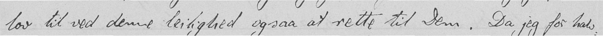lov til ved denne leilighed ogsaa at rette til Dem. Da jeg for halv-
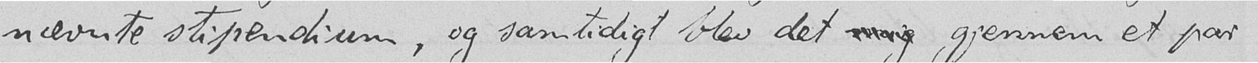nævnte stipendium, og samtidigt blev det  gjennem et par
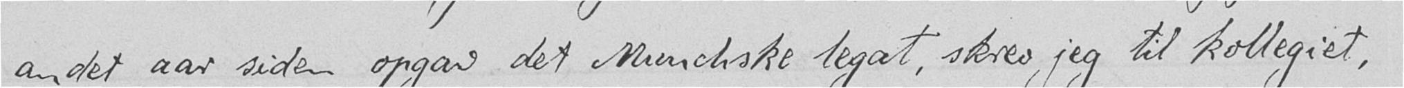andet aar siden opgav det Munchske legat, skrev jeg til kollegiet,
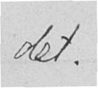det.
